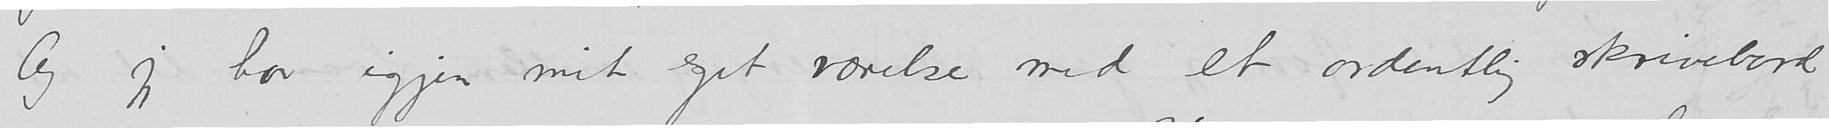Og jeg har igjen mit eget værelse med et ordentlig skrivebord
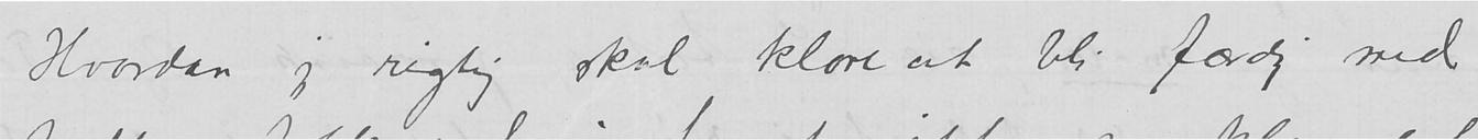Hvordan jeg rigtig skal klare at bli færdig med
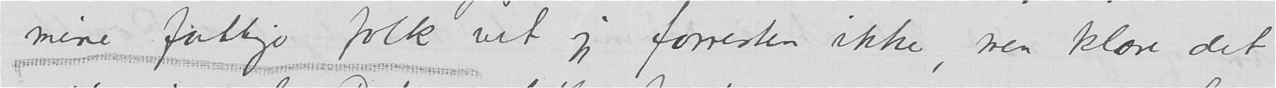mine fattige folk vet jeg forresten ikke, men klare det
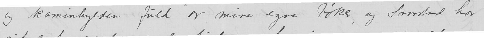og kaminhylden fuld av mine egne bøker, og Svarstad har
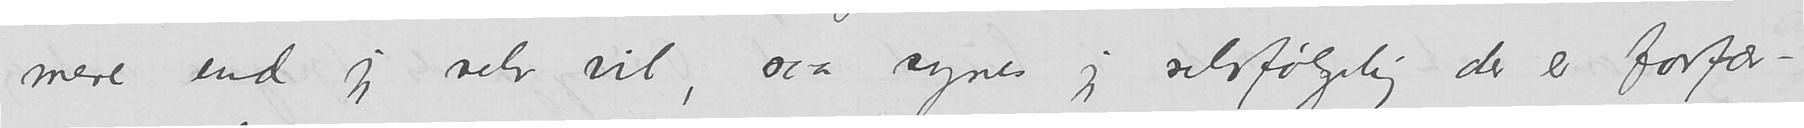mere end jeg selv vil, saa synes jeg selvfølgelig der er forfær-
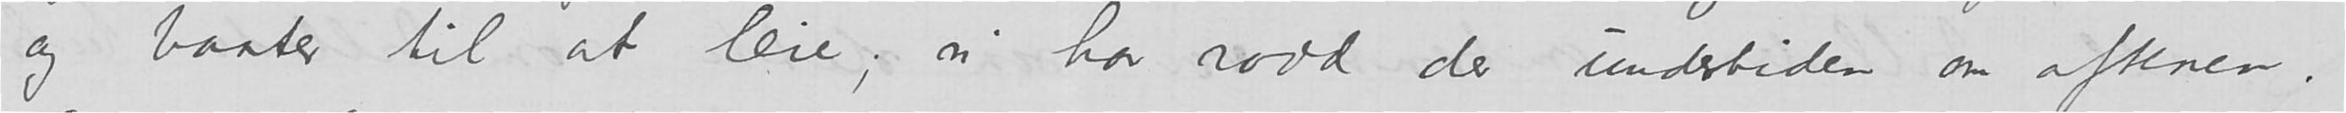og baater til at leie; vi har rood der undertiden om aftenen.
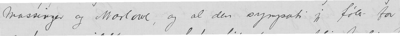Massinger og Marlowe, og al den sympati jeg føler for
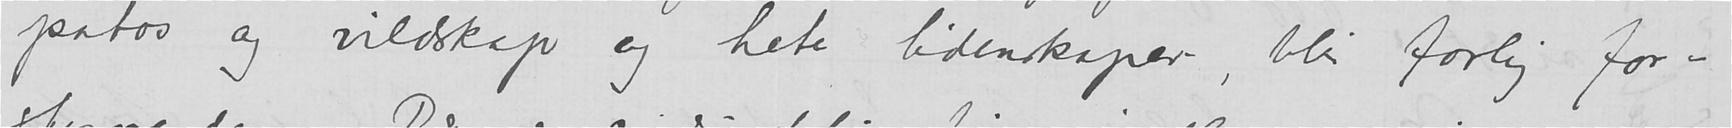patos og vildskap og hete lidenskaper, blir farlig for-
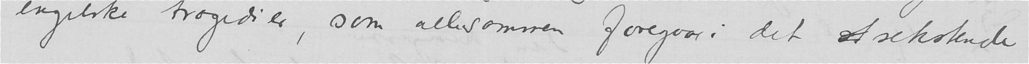engelske tragedier, som allesammen foregaar i det st sekstende
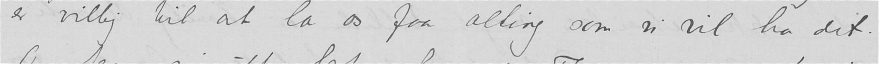er villig til at la os faa alting som vi vil ha det.
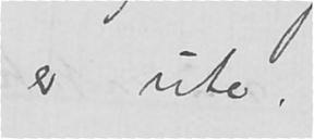vi er ute.
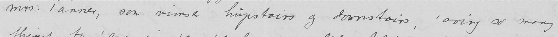mrs. Tanner, som vimser hupstairs og downstairs, ’aving so many
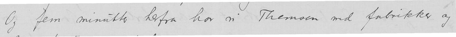Og fem minutter herfra har vi Themsen med fabrikker og
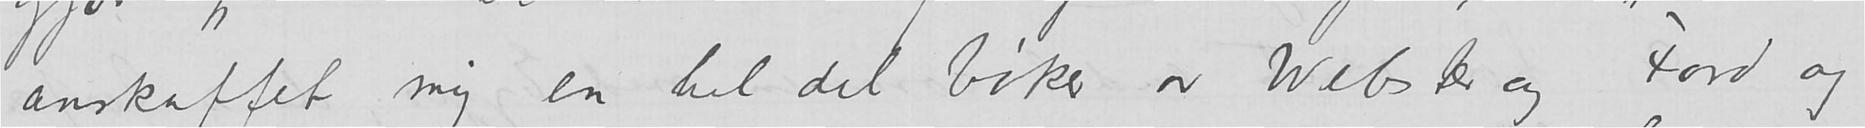anskaffet mig en hel del bøker av Webster og Ford og
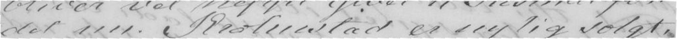det nu. Krohnstad er nylig solgt,
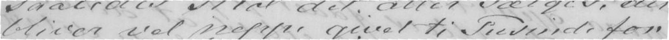bliver vel neppe givet ti Tusinde for
